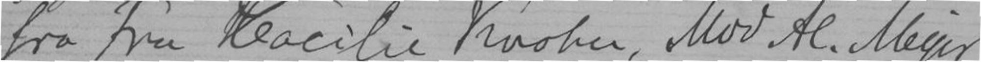fra Fru Cecilie Krohn, Mod Al. Meyer
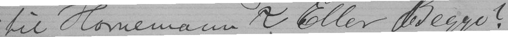til Horneman? Eller Begge?
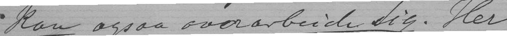kan ogsaa overarbeide sig. Her
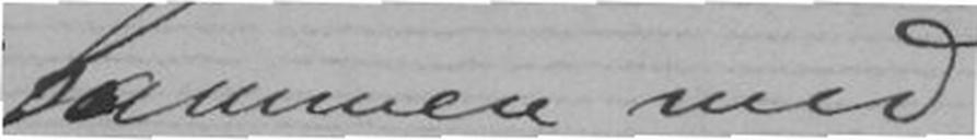sammen med
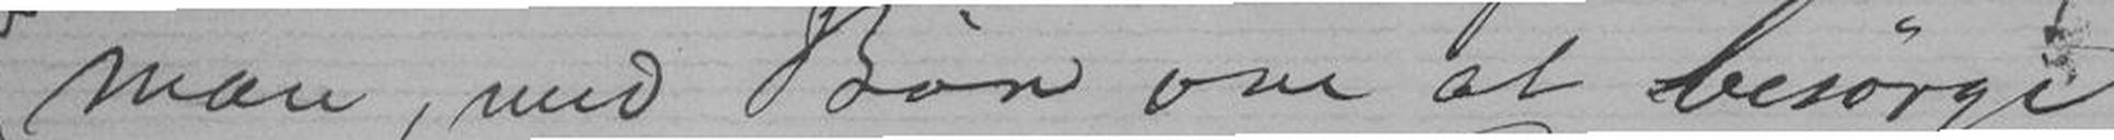man, med Bøn om at besørge
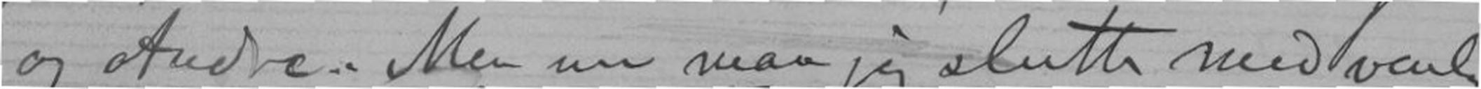og Andre. Men nu maa jeg slutte med venlig
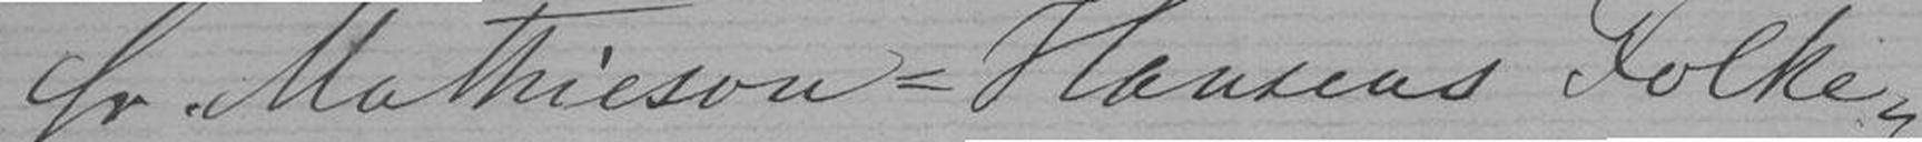hr. Mathieson-Hansens Folke-
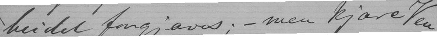beidet forgjeves; - men kjære Ven,
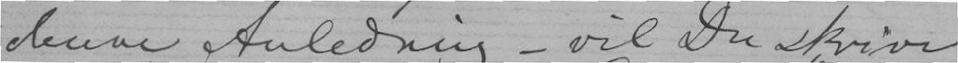i denne Anledning - vil Du skive
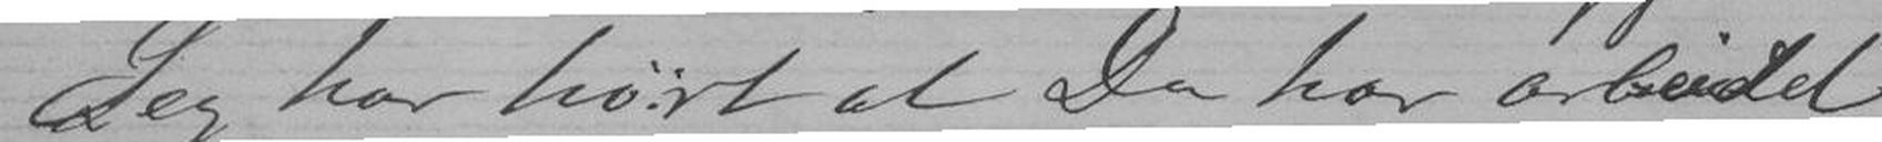Jeg har hørt at Du har arbeidet
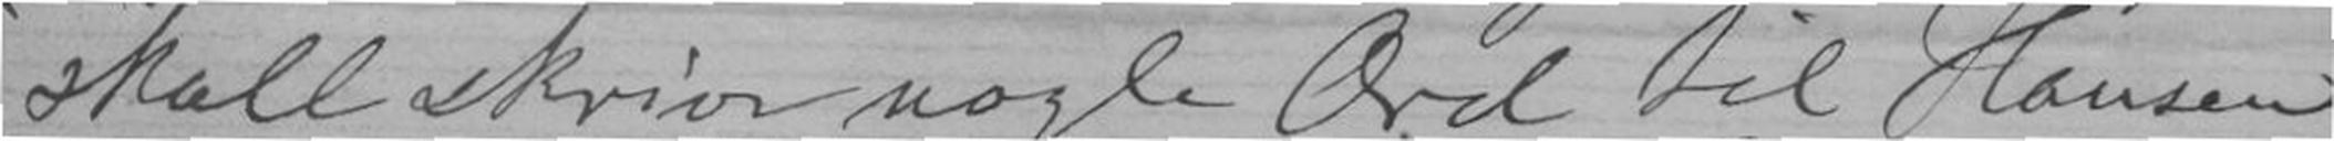skall skrive nogle Ord til Hansen
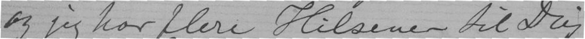og jeg har flere Hilsener til Dig
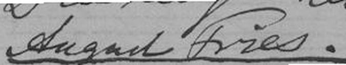August Fries.
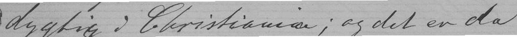dygtig i Christiania; og det er da
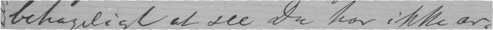behageligt at see Du har ikke ar-
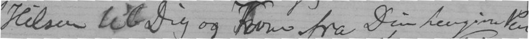Hilsen til Dig og Kone fra Din hengivne Ven
